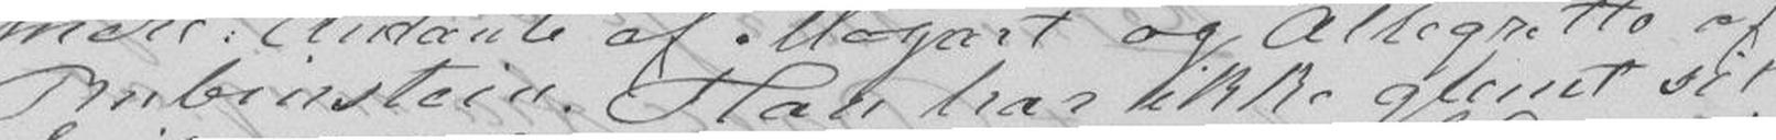Rubinstein. Han har ikke glemt sit
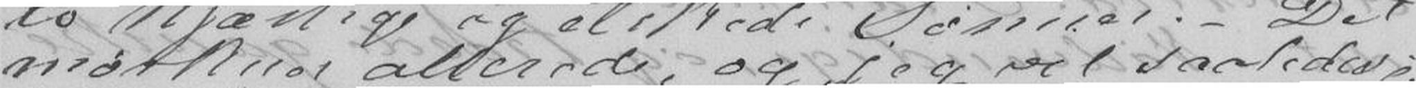mørkner allerede, og jeg vil saaledes ei
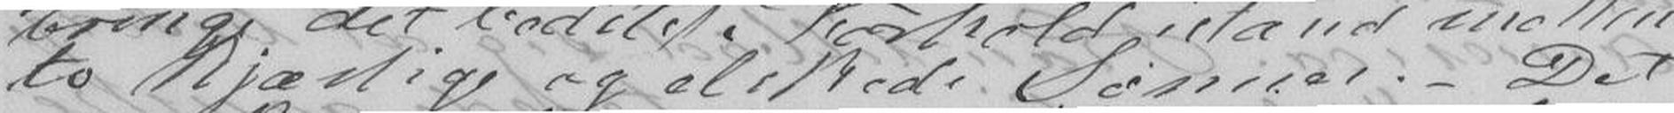to kjærlige og elskede Sønner. - Det
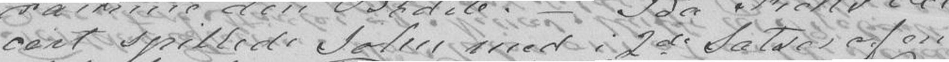cert spillede John med i 2de Satse at en
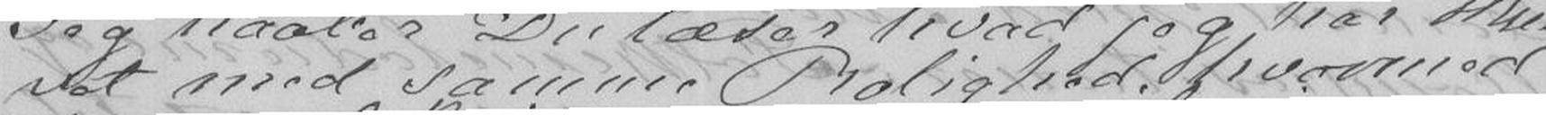vet med samme Rolighed hvormed
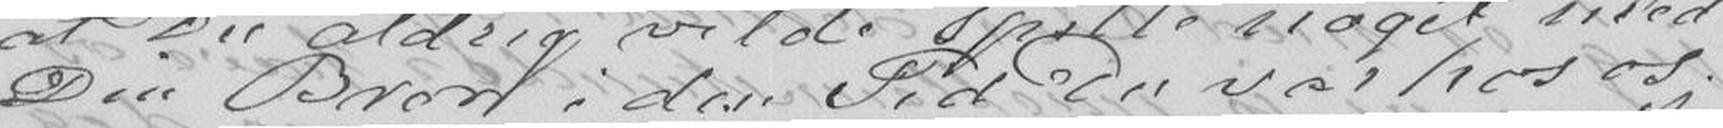Din Bror i den Tid Du var hos os.
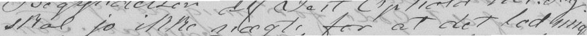skal jo ikke nægte for at det lod mig
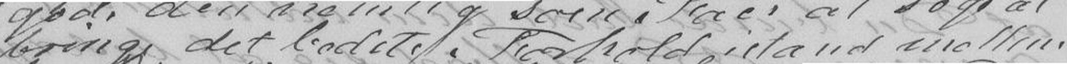bringe det bedste Forhold istand mellem
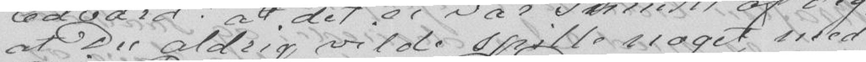at Du aldrig vilde spille noget med
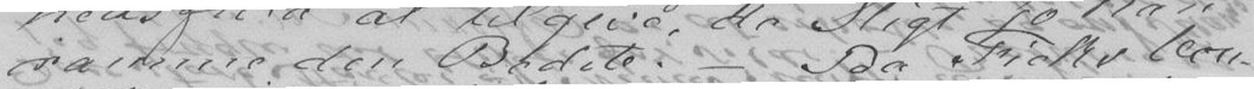ramme den Bedste. - Paa Ficks Con-
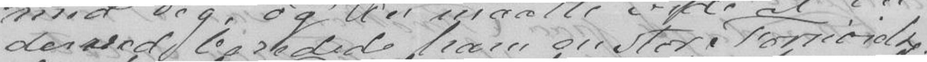derved beredede ham en stor Fornøielse.
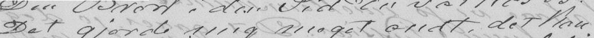Det gjorde mig meget ondt, det kan
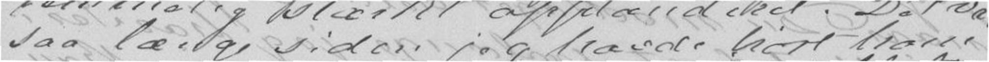saa længe siden jeg havde hørt ham
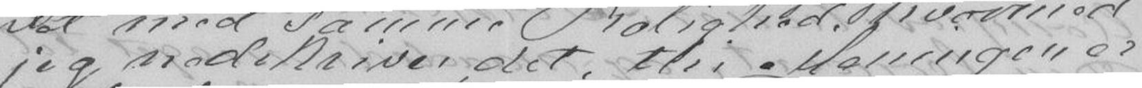jeg nedskriver det; thi Meningen er
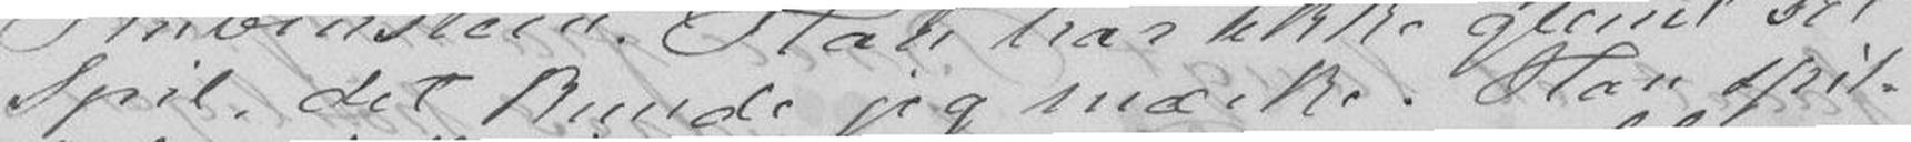Spil, det kunde jeg mærke. Han spil-
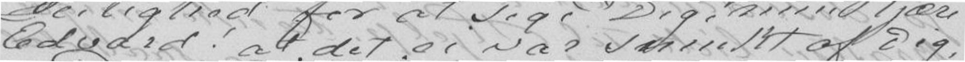Edvard! at det ei var smukt af Dig
